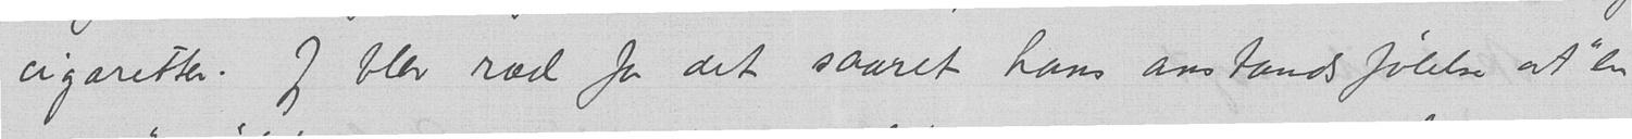cigaretter. Jeg blev ræd for det saaret hans anstandsfølelse at "en
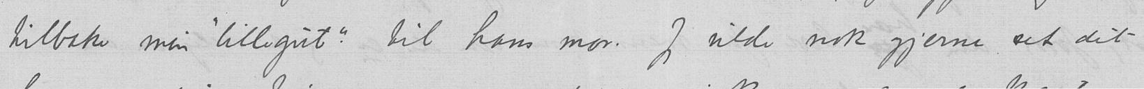tilbake min "lillegut" til hans mor. Jeg vilde nok gjerne set dit
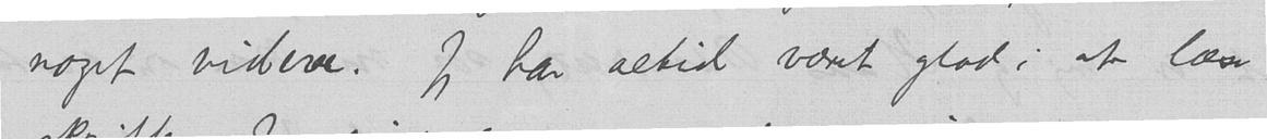noget videre. Jeg har altid været glad i at læse
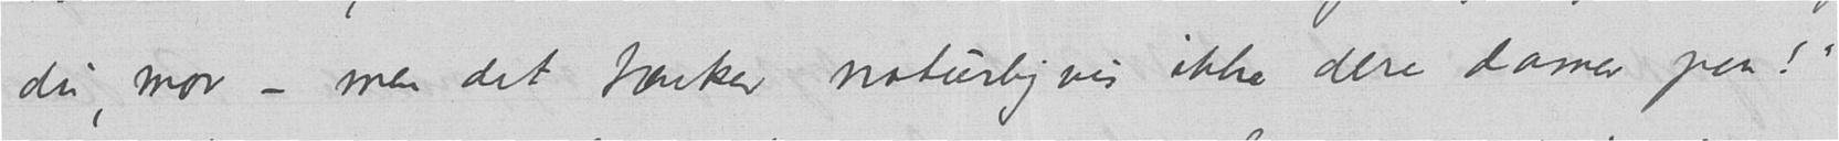du, mor - men det tænker naturligvis ikke dere damer paa!"
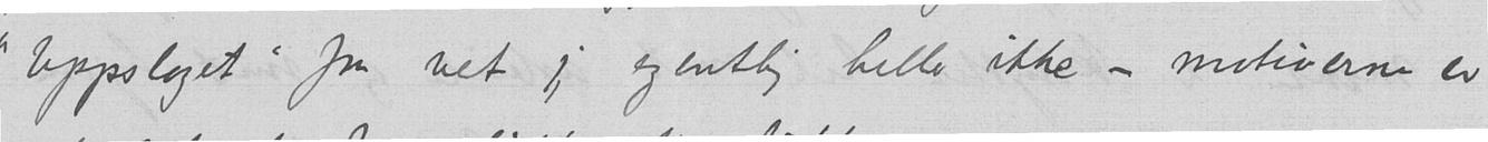"uppslaget" fra vet jeg egentlig heller ikke - motiverne er
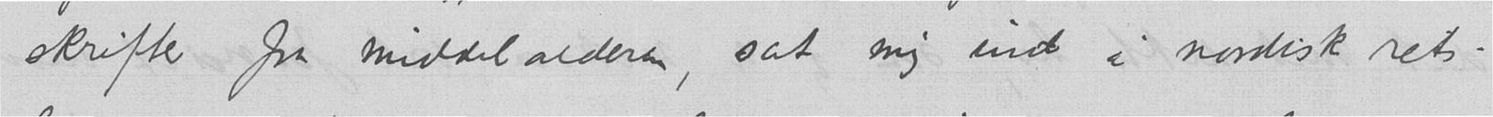skrifter fra middelalderen, sat mig ind i nordisk rets-
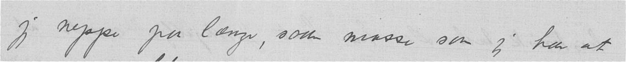jeg neppe paa længe, saa masse som jeg har at
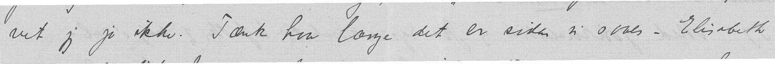vet jeg jo ikke. Tænk hvor længe det er siden vi saaes - Elisabeth
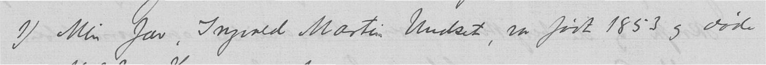1) Min far, Ingvald Martin Undset, var født 1853 og døde
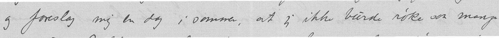og foreslog mig en dag i sommer, at jeg ikke burde røke saa mange
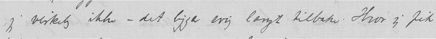jeg virkelig ikke - det ligger evig langt tilbake. Hvor jeg fik
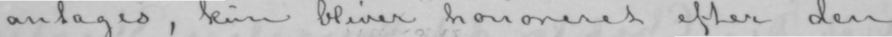antages, kun bliver honoreret efter den
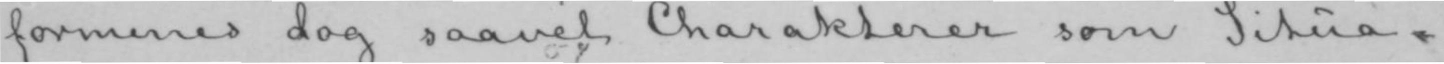formenes dog saavel Charakterer som Situa-
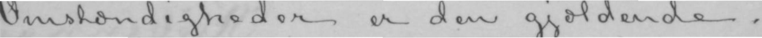Omstændigheder er den gjældende.
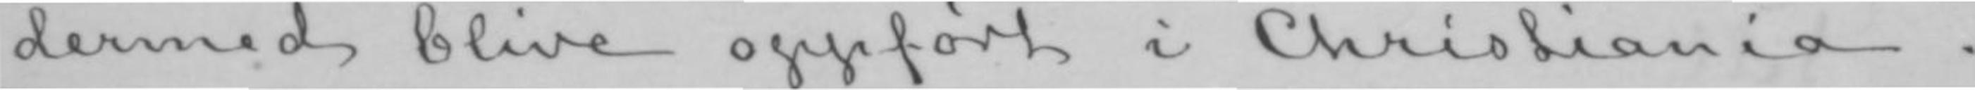dermed blive oppført i Christiania.
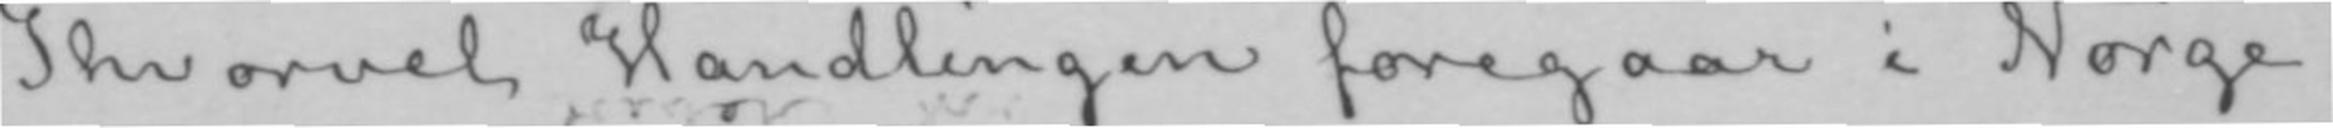Ihvorvel Handlingen foregaar i Norge,
